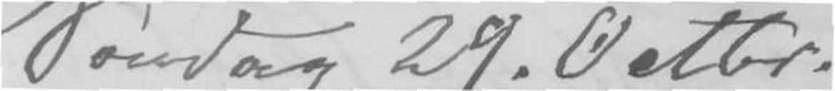Søndag 29. Octbr
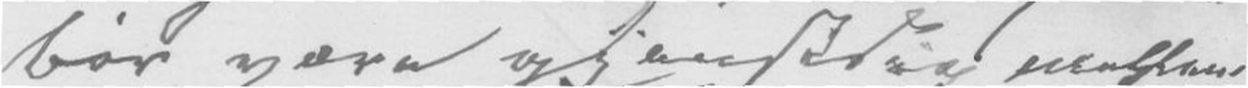bør være gjensidig mellem
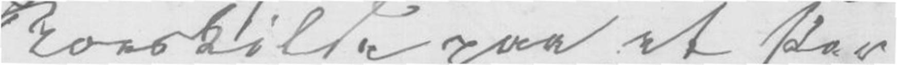Roeskilde paa et Par
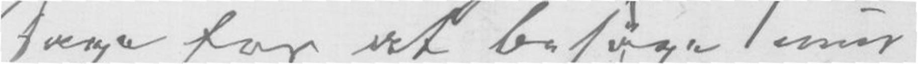Dage for at besøge min
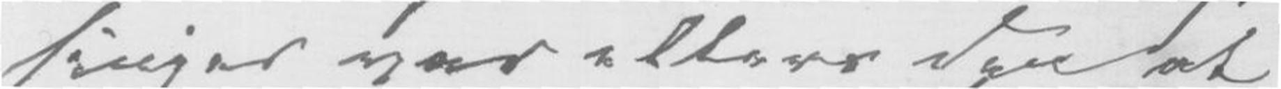Linjer var ellers den at
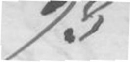93
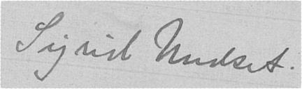Sigrid Undset.
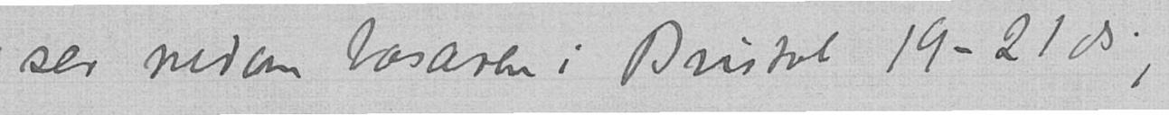ser nedom basaren i Bristol 19-21 ds.,
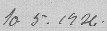10.5.1926.
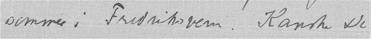sommer i Fredriksvern. Kanske De
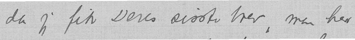da jeg fik Deres sisste brev, men her
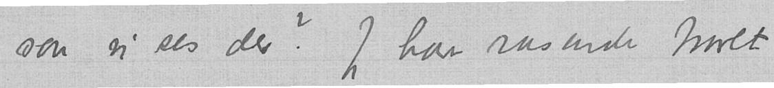saa vi ses der? Jeg har rasende travlt
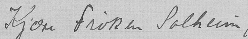Kjære Frøken Solheim,
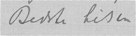Bedste hilsen
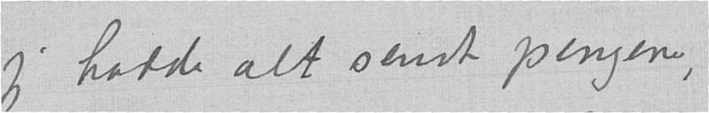jeg hadde alt sendt pengene,
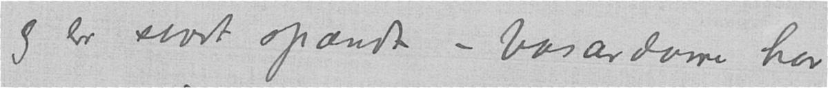og er svært spændt – basardame har
